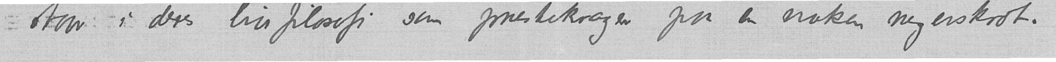staar i deres livsfilosofi som prestekrager paa en naken negerskrot.
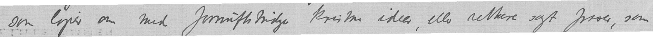som løper om med fornuftstridige kristne ideer, eller rettere sagt fraser, som
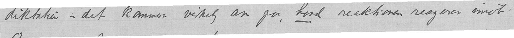diktatur – det kommer virkelig an paa, hvad reaktionen reagerer imot.
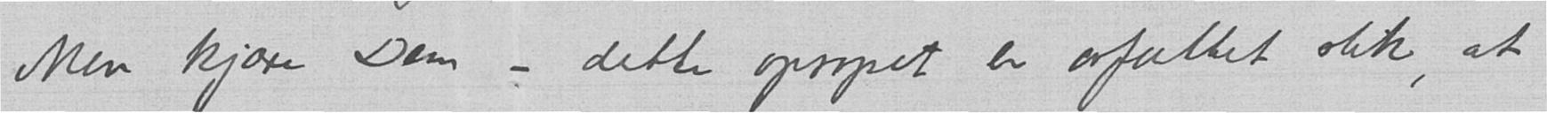Men kjære Dem – dette opropet er avfattet slik, at
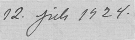12. juli 1924.
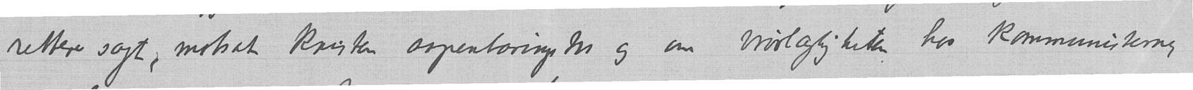rettere sagt; motsat kristen aapenbaringslov og om vrøvlagtigheten hos kommunisterne,
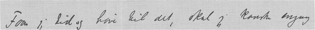Faar jeg tid og høve til det, skal jeg kanske engang
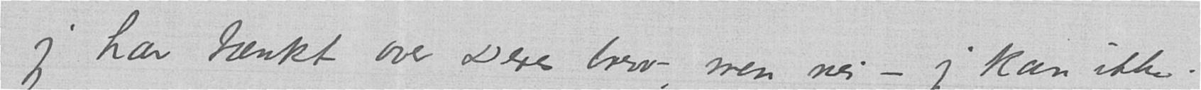jeg har tænkt over Deres brev, men nei – jeg kan ikke.
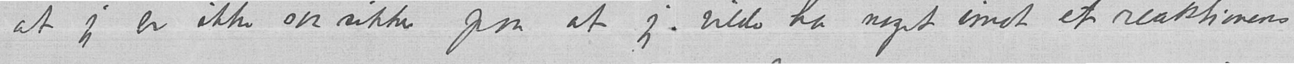at jeg er ikke saa sikker paa at jeg vilde ha noget imot et reaktionens
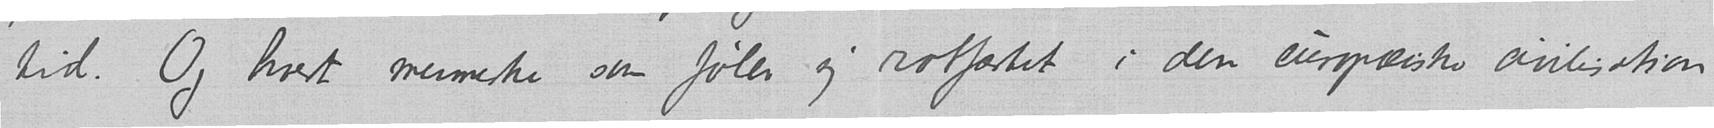tid. Og hvert menneske som føler sig rotfæstet i den europeiske civilisation
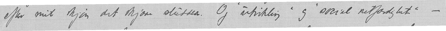efter mit skjøn det skjære sludder. Og "utvikling" og "social retfærdighet» –
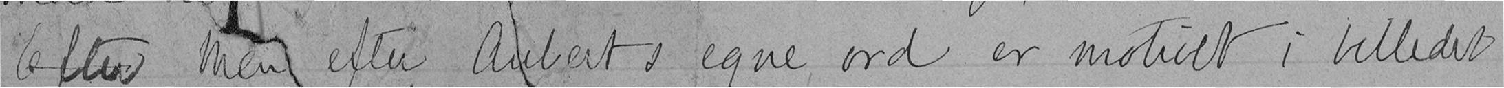Efter Men efter Auberts egne ord er motivet i billedet
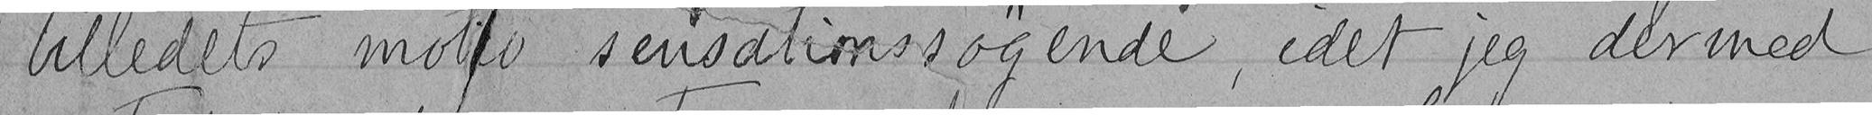billedets motiv sensationssøgende, idet jeg dermed
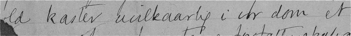ld kaster uvilkaarlig i vor dom, et
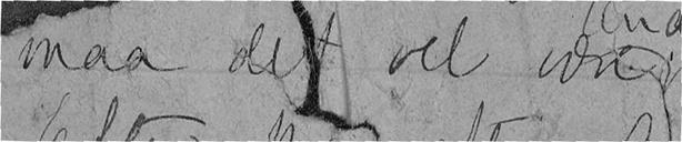maa det vel være
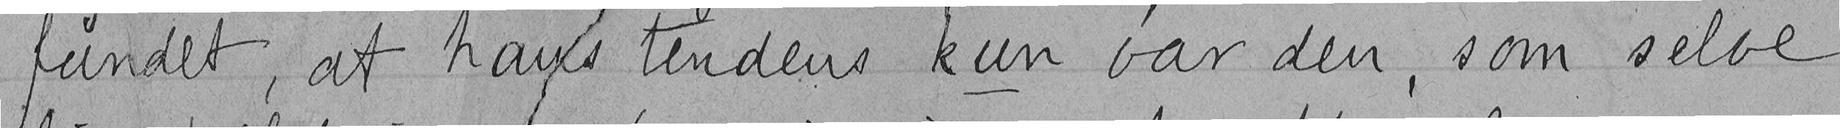fundet, at hans tendens kun var den, som selve
